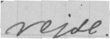rejse.
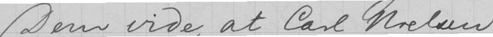Dem vide, at Carl Nielsen
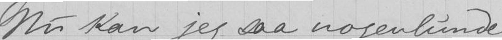Nu kan jeg saa nogenlunde
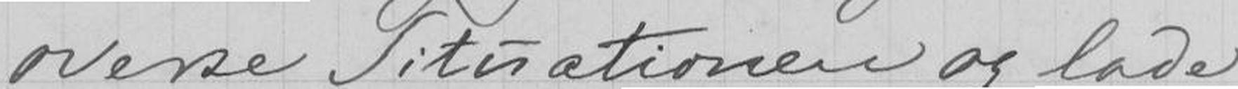overse Situationen og lade
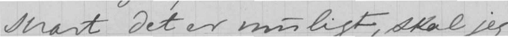snart det er muligt, skal jeg
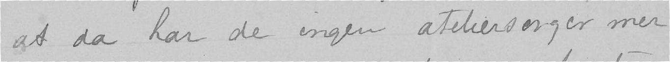at da har de ingen ateliersorger mer
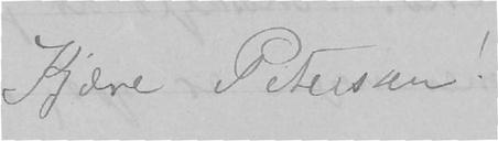Kjære Peterssen!
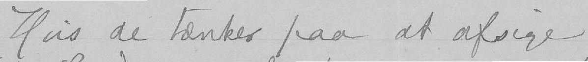Hvis de tænker paa at afsige
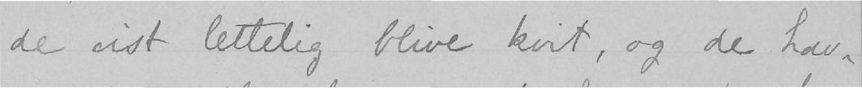de vist lettelig blive kvit, og de hav-
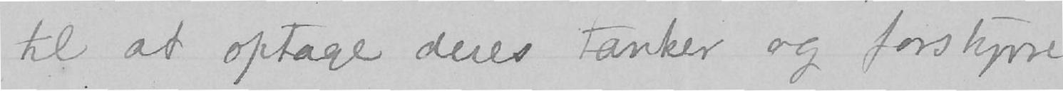til at optage deres tanker og forstyrre
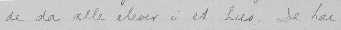de da alle elever i et hus. De har
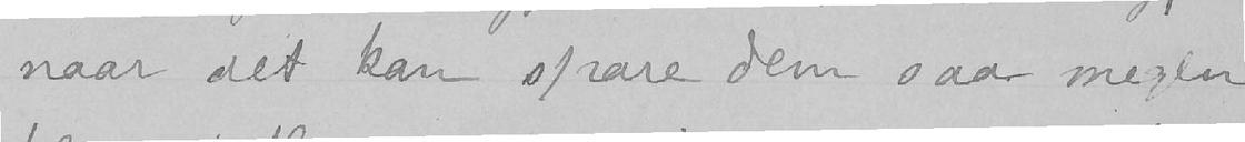naar det kan spare dem saa megen
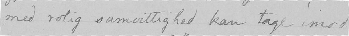med rolig samvittighed kan tage imod
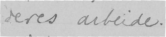deres arbeide.
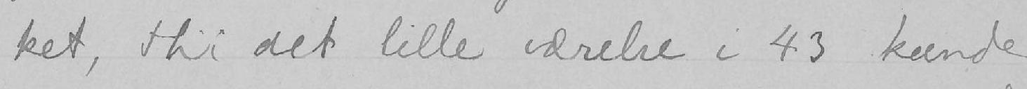ket, thi det lille værelse i 43 kunde
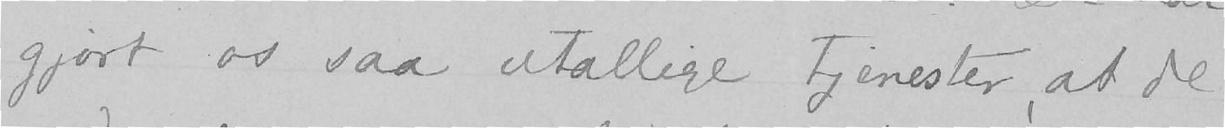gjort os saa utallige tjenester, at de
